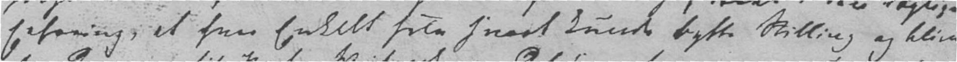Erfaring; at hver Enkelt selv snart kunde bytte Stilling og blive
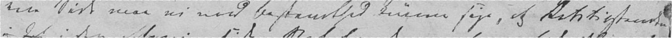ene Side maa vi med Bestemthed kunne sige, at Retstilstanden
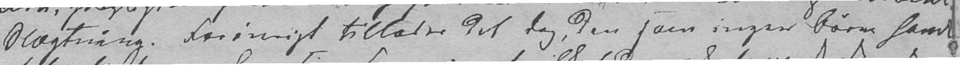Slægtning. Forøvrigt tillodes det dog, den som ingen Børn havde
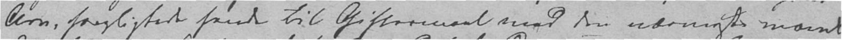Arv, forpligtede hende til Giftermaal med den nærmeste mandl.
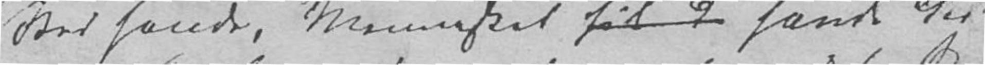Sted havde, Mennesket havde der
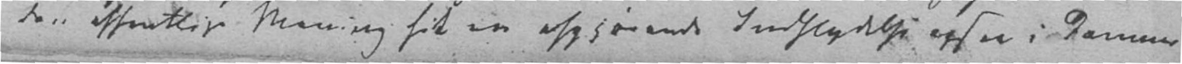den offentlige Mening fik en afgjørende Indflydelse ogsaa i Dommer
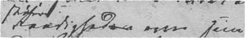Raadighed over sine
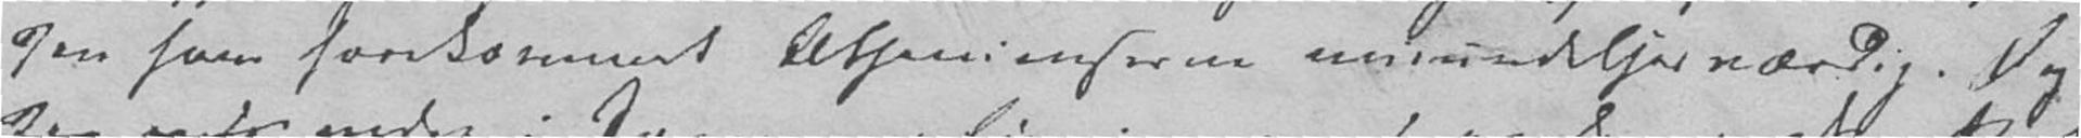den have forekommet Athenienserne misundelsesværdig. Og
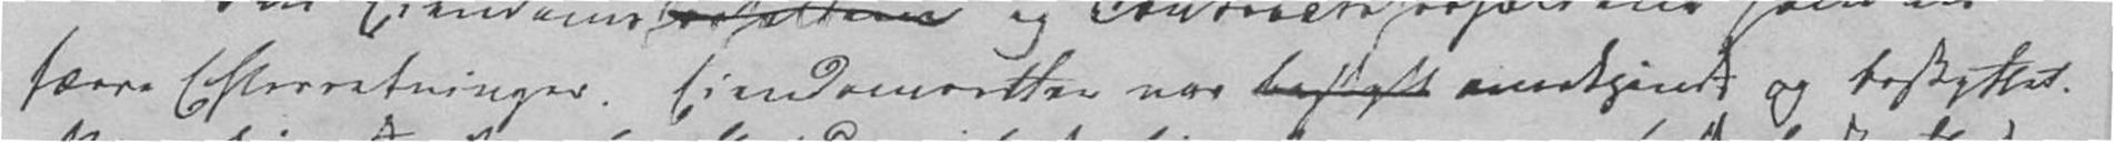færre Efterretninger. Eiendomsretten var anerkjendt og beskyttet.
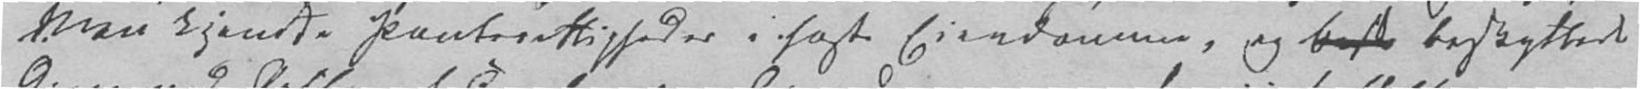Man kjendte Panterettigheder i faste Eiendomme, og beskyttede
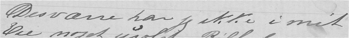Desværre har jeg ikke i mit
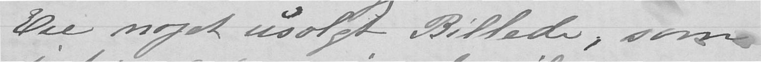Eie noget usolgt Billede, som
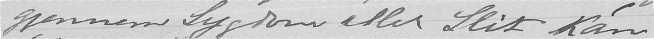gjennem Sygdom ellet Slit kan
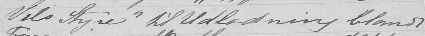Vels Styre" til Udlodning blandt
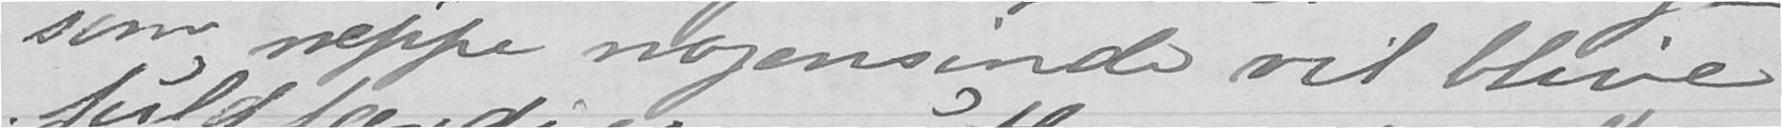som neppe nogensinde vil blive
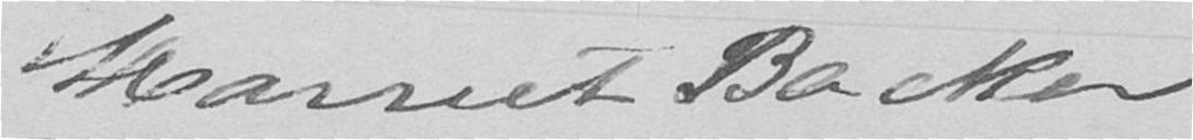Harriet Backer
